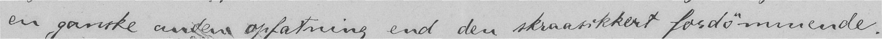en ganske anden opfatning end den skraasikkert fordømmende.
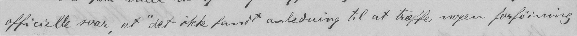officielle svar, at "det ikke fandt anledning til at træffe nogen forføining
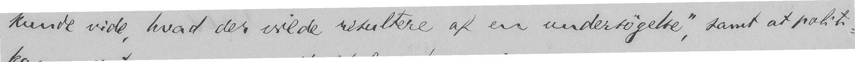kunde vide, hvad der vilde resultere af en undersøgelse", samt at politi-
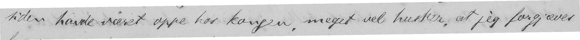siden havde været oppe hos kongen, meget vel husker, at jeg forgjæves
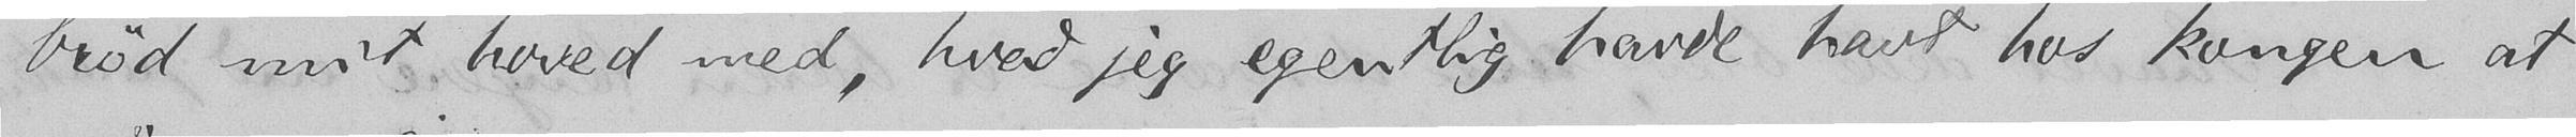brød mit hoved med, hvad jeg egentlig havde havt hos kongen at
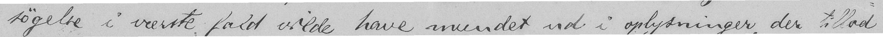søgelse i værste fald vilde have mundet ud i oplysninger, der tillod
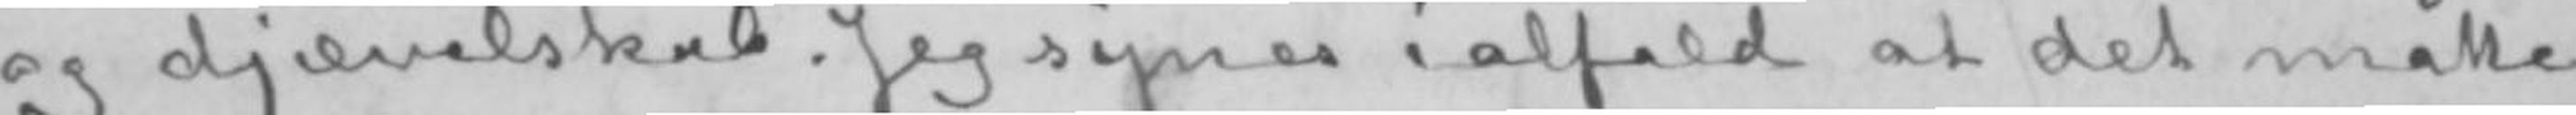og djævelskab. Jeg synes ialfald at det måtte
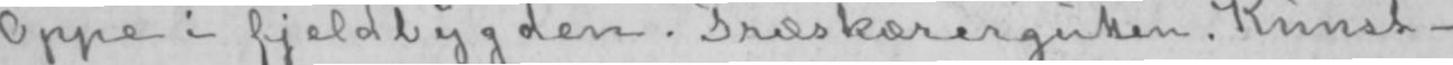Oppe i fjeldbygden. Træskærergutten. Kunst-
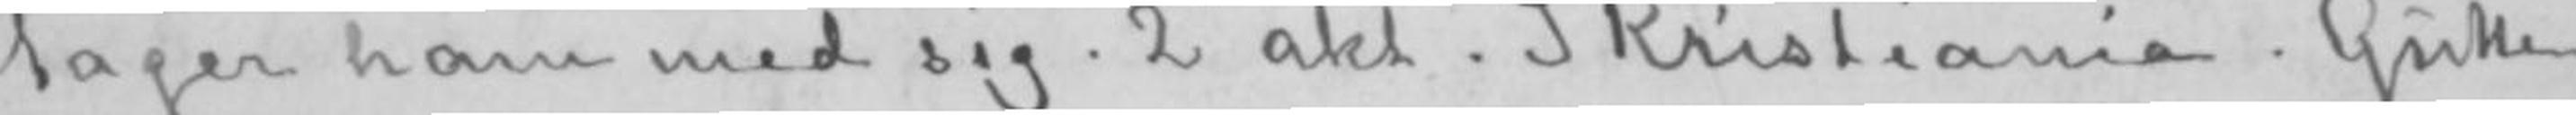tager ham med sig. 2de akt. I Kristiania. Gutten
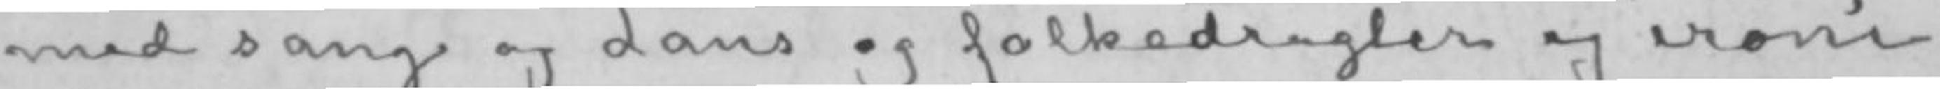med sang og dans og folkedragter og ironi
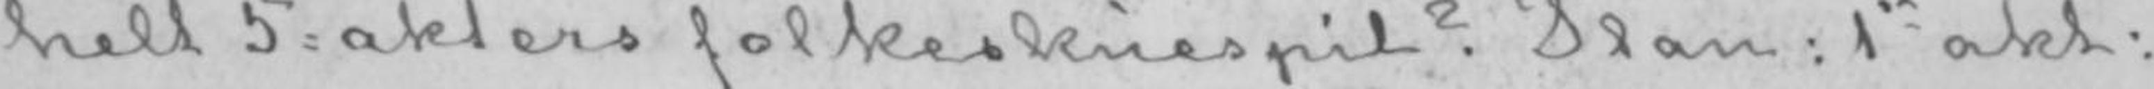helt 5-akters folkeskuespil? Plan: 1ste akt:
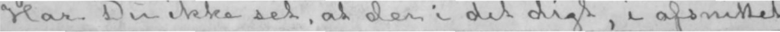Har Du ikke set, at der i dit digt, i afsnittet
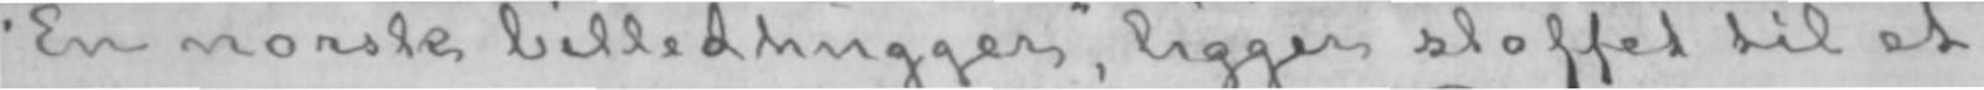«En norsk billedhugger», ligger stoffet til et
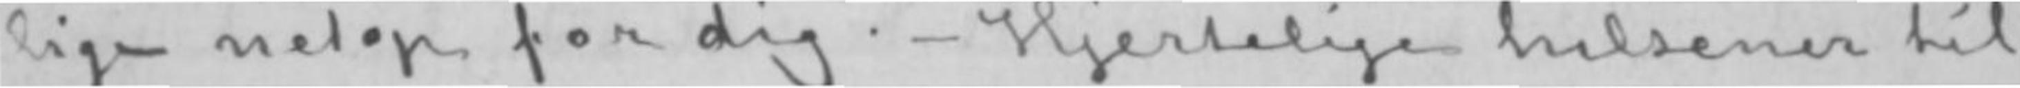lige netop for dig.- Hjertelige hilsener til
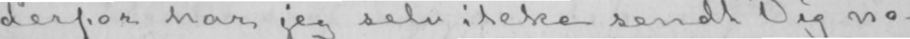derfor har jeg selv ikke sendt Dig no-
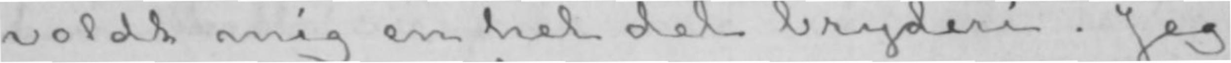voldt mig en hel del bryderi. Jeg
